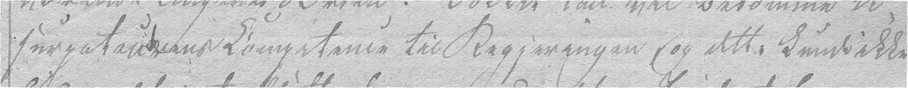surpateurens Competence til Regjeringen og dette kunde ikke
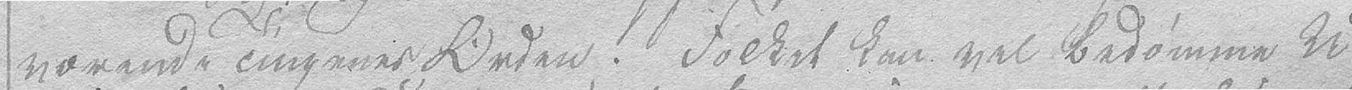værende Tingenes Orden. Folket kan vel bedømme U-
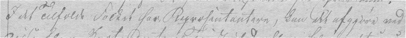I det Tilfælde Folket har Repræsentantere, kan det afgjøre ved
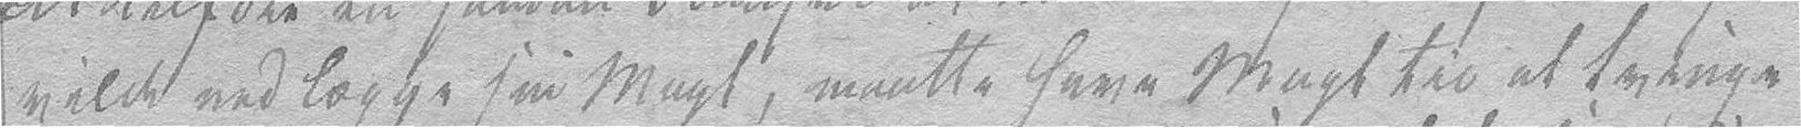vilde nedlægge sin Magt, maatte have Magt til at tvinge
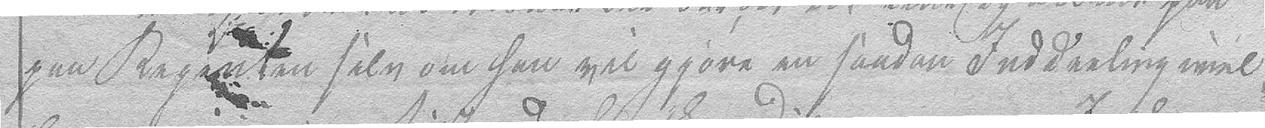paa Regenten selv om han vil gjøre en saadan Inddeeling imel-
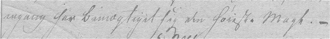engang har bemægtiget sig den høieste Magt. –
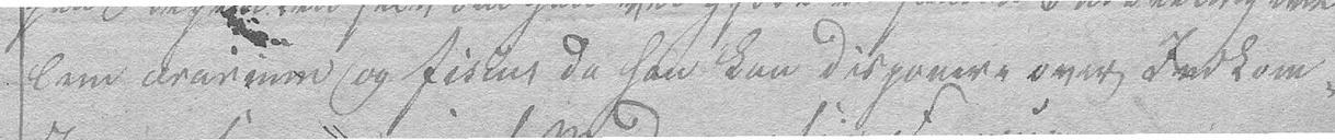lem ærarium og fiscus da han kan disponere over Indkom-
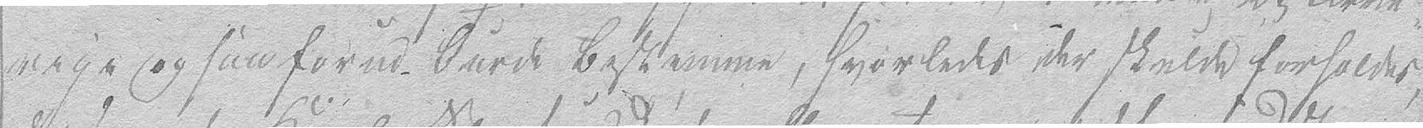rige ogsaa forud kunde bestemme, hvorledes der skulde forholdes
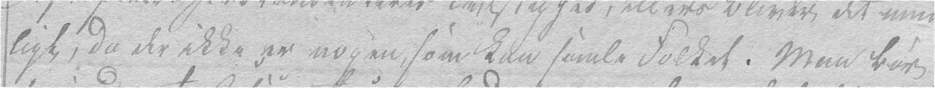ligt, da der ikke er nogen som kan samle Folket. Man bør
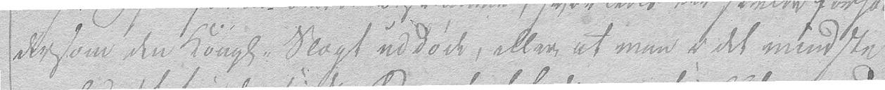dersom den Kongl. Slægt uddøde, eller at man i det mindste
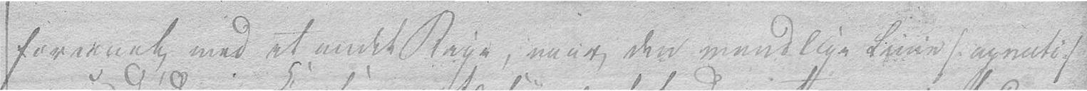foreenet med et andet Rige, naar den mandlige Linie /: agnati :/
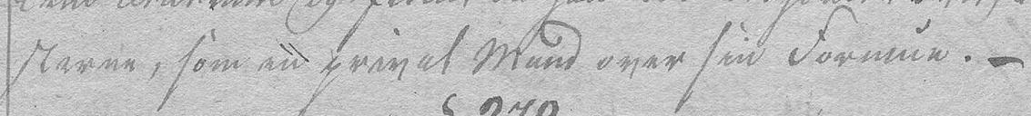sterne, som en privat Mand over sin Formue. –
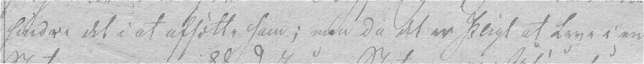hindre det i at afsætte ham; men da det er Pligt at leve i en
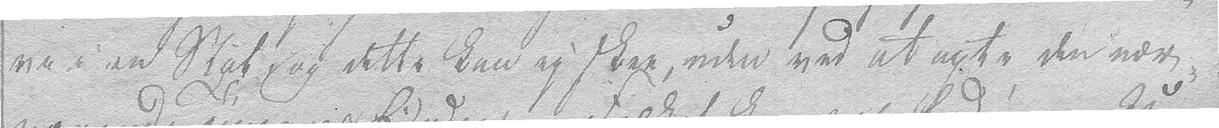ve i en Stat, og dette kan ey skee uden ved at agte den nær-
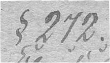§ 272.
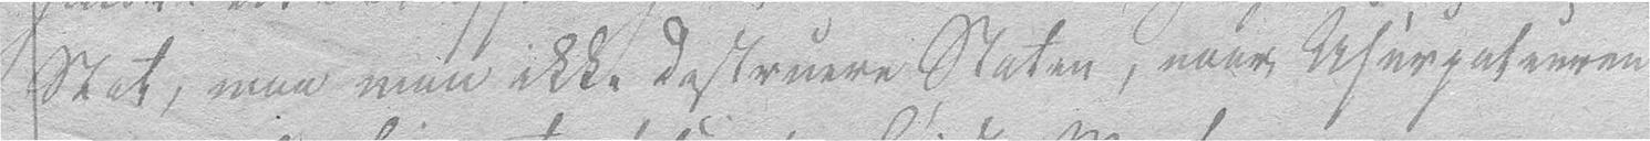Stat, maa man ikke destruere Staten, naar Usurpateuren
